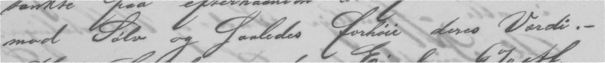mod Sølv og Saaledes forhøie deres Værdi. -
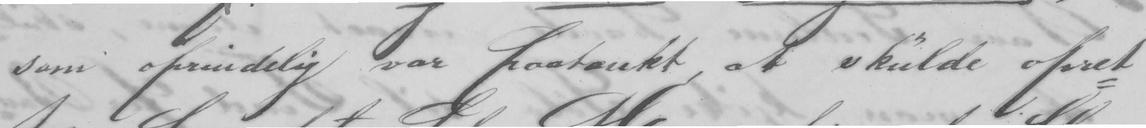som oprindelig var paatænkt, at skulde opret-
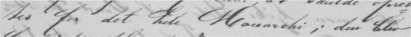tes for det hele Monarchi; i den blev
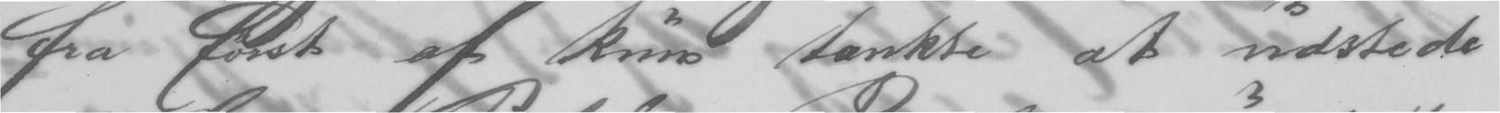fra først af kun Tænkte at udstede
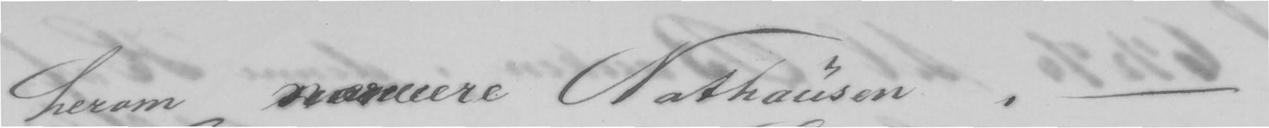herom nærmere Nathausen. -
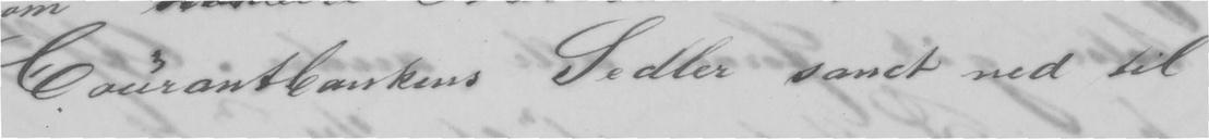Courantbankens Sedler samt ned til
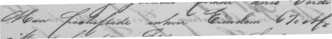Man paaheftede enhver Eiendom 6% Af-
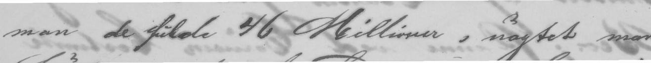man de fulde 46 Millioner, uagtet man
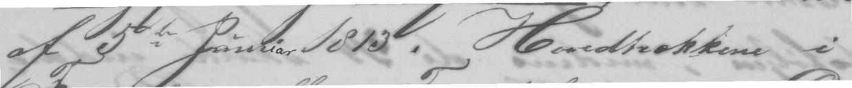af 5de Junuar 1813. Hovedtrækkene i
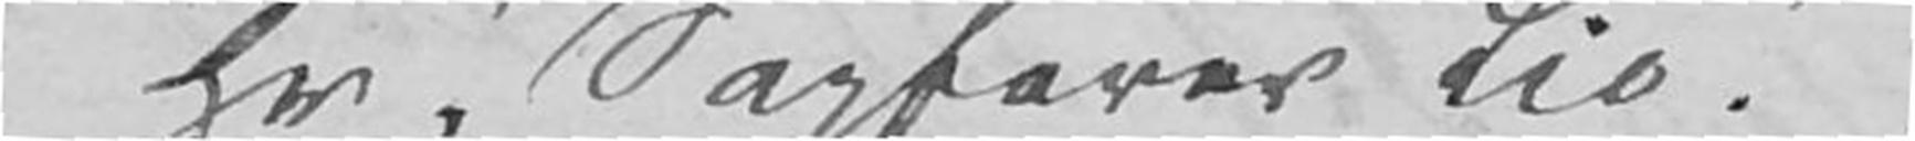Hr Sagfører Lie!
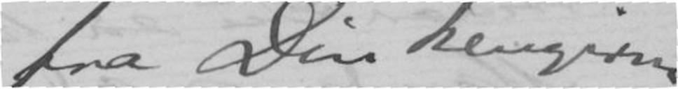fra Din hengivne
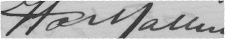Otto Malling
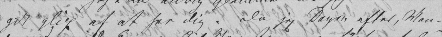gik glip af at see Dig. Da jeg Dagen efter, Man-
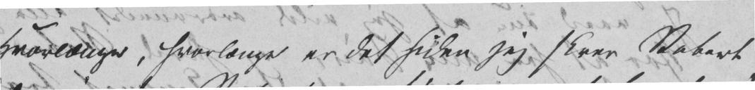Hvorlænge, hvorlænge er det siden jeg skrev Robert
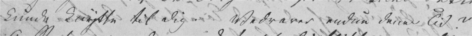kunde knytte til Dig. Vedvarer endnu denne Tid?
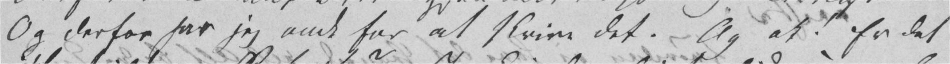Og derfor har jeg ondt for at skrive det. Og ak! Er det
